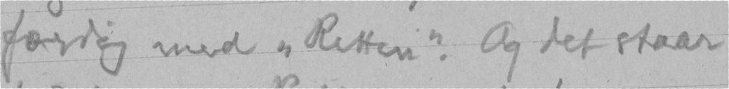færdig med "Retten". Og det staar
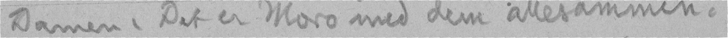Damen. Det er Moro med dem allesammen.
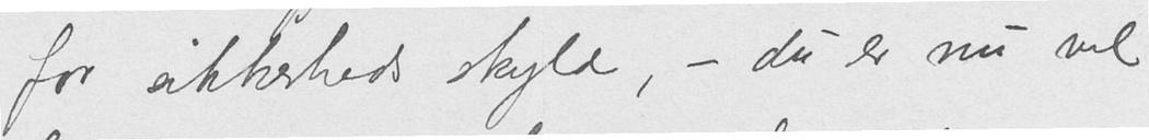for sikkerheds skyld, - du er nu vel
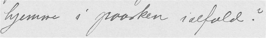hjemme i paasken ialfald?
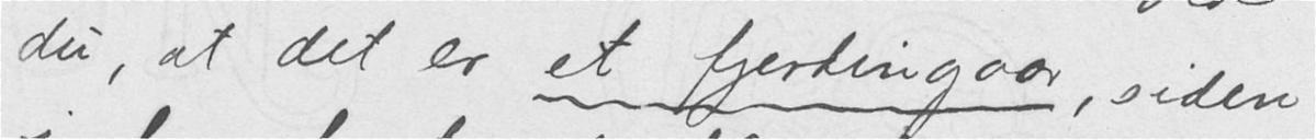du, at det er et fjerdingaar, siden
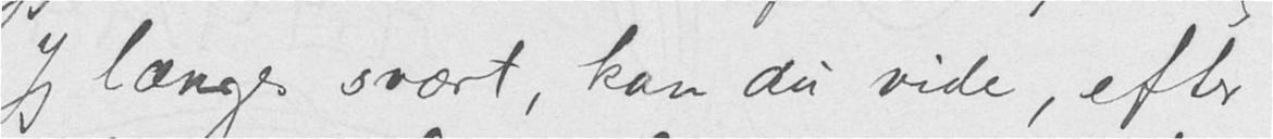Jeg længes svært, kan du vide, efter
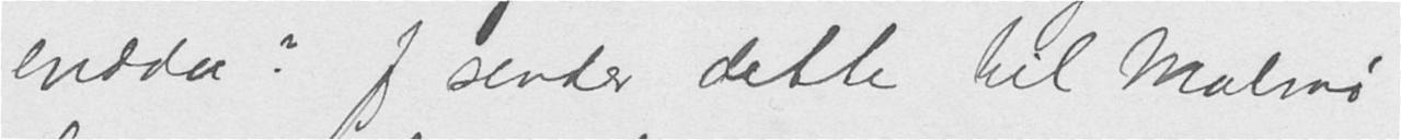endda? Jeg sender dette til Malmø
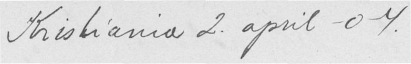Kristiania 2. april -04.
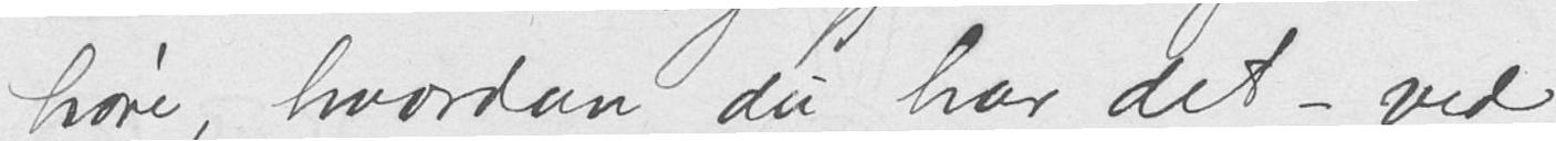høre, hvordan du har det - ved
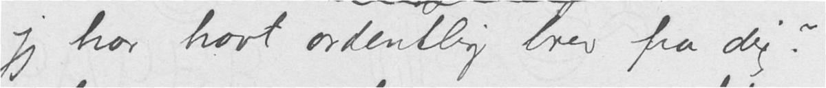jeg har havt ordentlig brev fra dig?
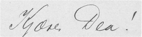Kjære Dea!
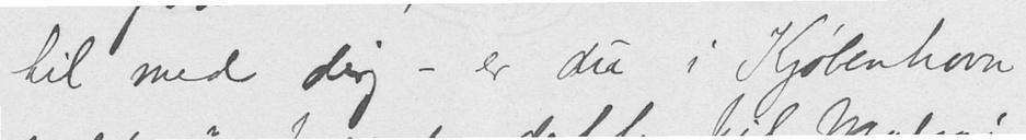til med dig - er du i Kjøbenhavn
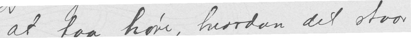at faa høre, hvordan det staar
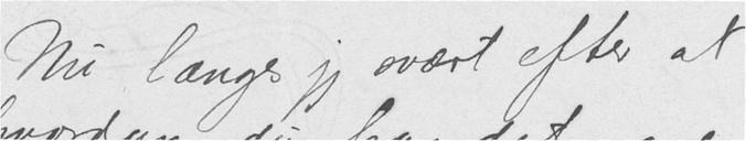Nu længes jeg svært efter at
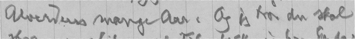Alverdens mange Aar. Og jeg tror du skal
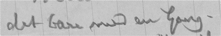det bare med en Gang.
